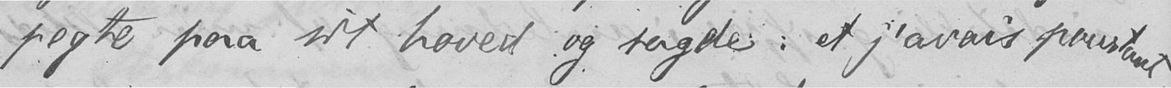pegte paa sit hoved og sagde: et j'avais pourtant
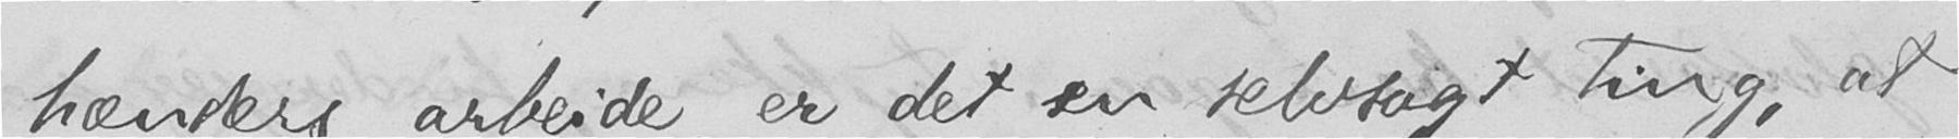hænders arbeide, er det en selvsagt ting, at
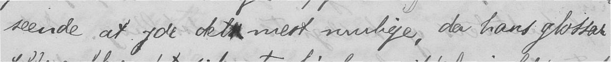seende at yde det mest mulige, da hans glossar
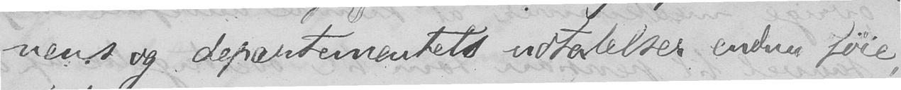nens og departementets udtalelser endnu føie
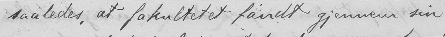saaledes, at fakultetet fandt gjennem sin
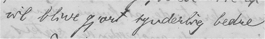vil blive gjort synderlig bedre.
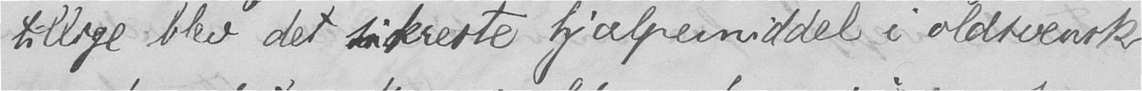tillige blev det sikreste hjælpemiddel i oldsvensk
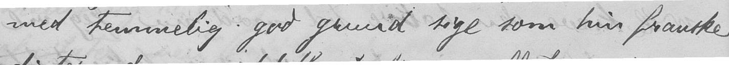med temmelig god grund sige som hin franske
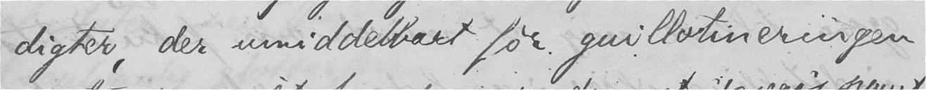digter, der umiddelbart før guillotineringen
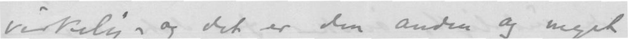virkelig og det er den anden og meget
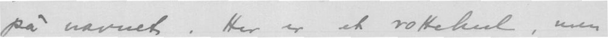på navnet. Her er et rottehul, men
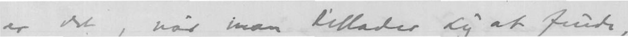er det, når man tillader sig at finde,
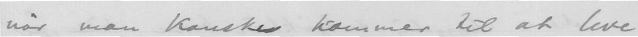når man kanske kommer til at leve
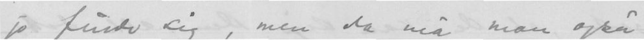jo finde sig, men da må man også
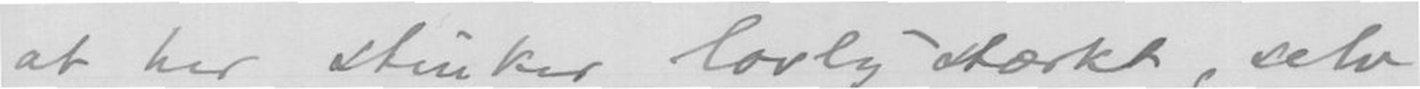at her stinker lovlig stærkt, selv
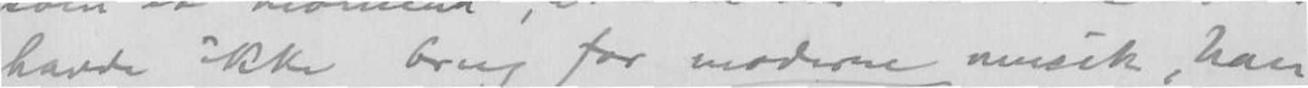havde ikke brug for moderne musik, han
